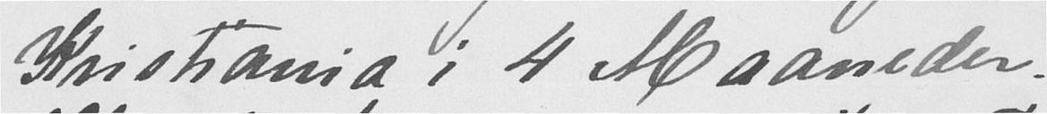Kristiania i 4 Maaneder.
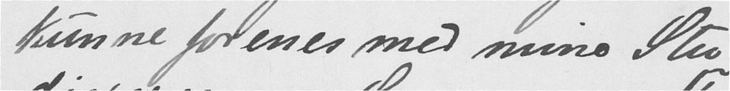kunne forenes med mine Stu-
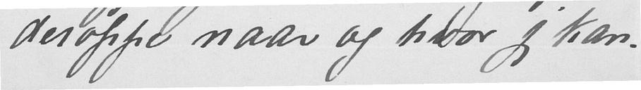deroppe naar og hvor jeg kan.
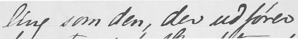ling som den, der udfører
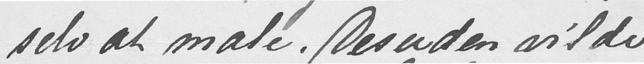selv at male. Desuden vilde
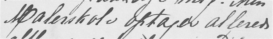Malerskole optager allerede
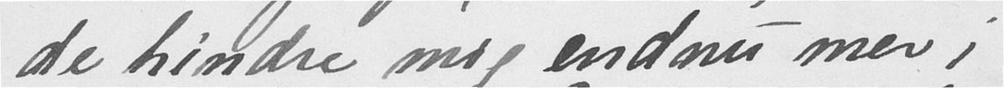de hindre mig endnu mer i
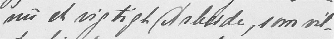nu et vigtigt Arbeide, som vil-
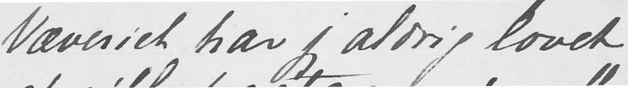Væveriet har jeg aldrig lovet
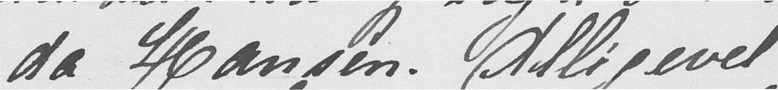da Hansen. Alligevel
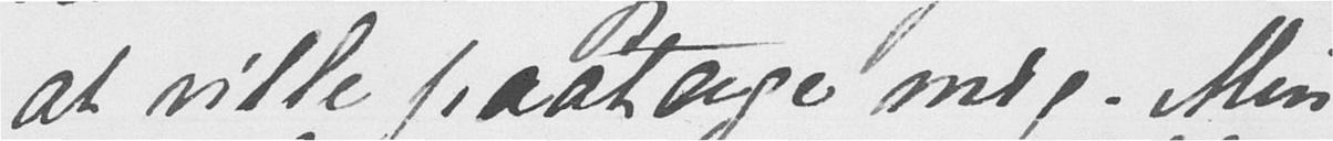at ville paatage mig. Min
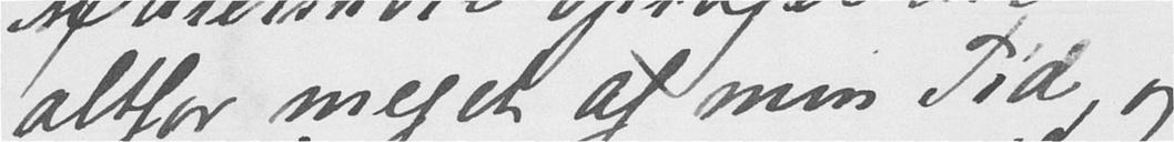altfor meget af min Tid, og
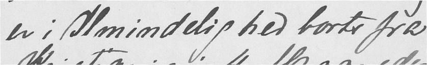er i Almindelighed borte fra
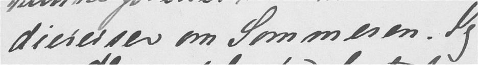diereiser om Sommeren. Jeg
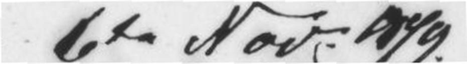6te Nov. 1879.
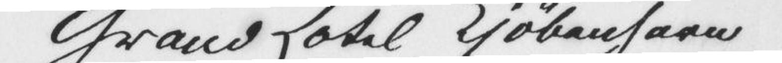Grand Hotel Kjøbenhavn
